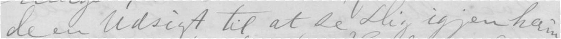de en Udsigt til at se Dig igjen herin-
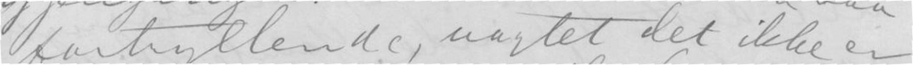fortryllende, uagtet det ikke er
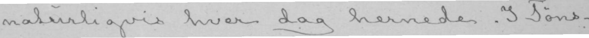naturligvis hver dag hernede. I Tøns-
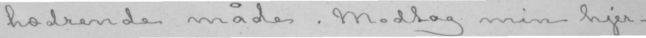hædrende måde. Modtag min hjer-
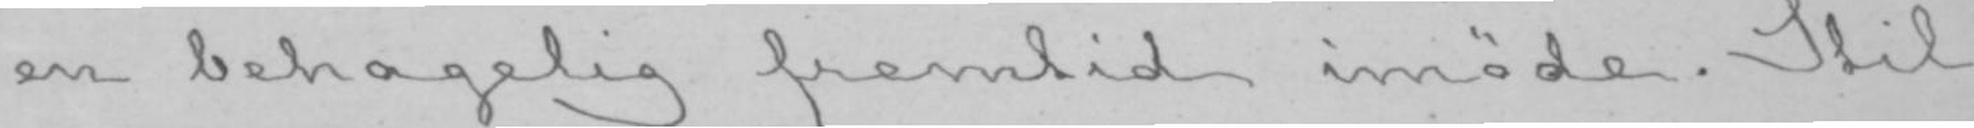en behagelig fremtid imøde. Stil-
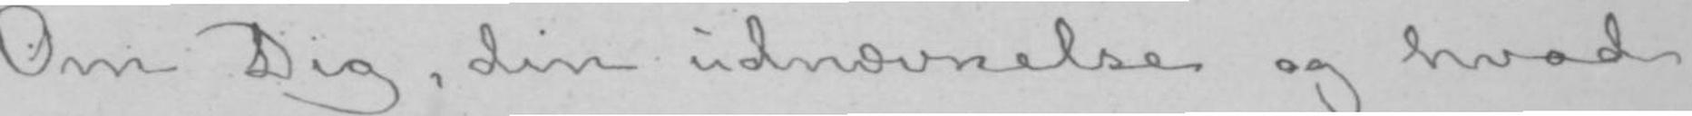Om Dig, din udnævnelse og hvad
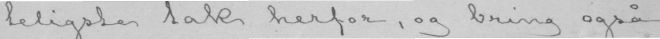teligste tak herfor, og bring også
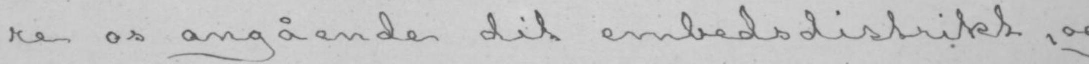re os angående dit embedsdistrikt, og
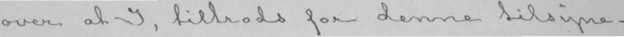over at I, tiltrods for denne tilsyne-
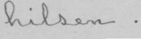hilsen.
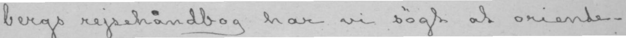bergs rejsehåndbog har vi søgt at oriente-
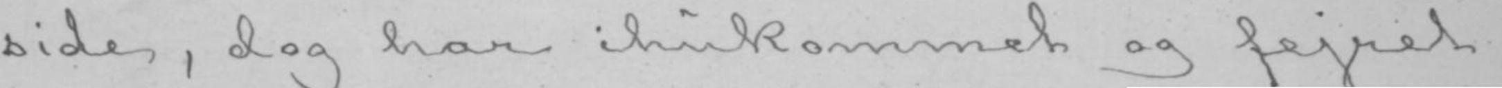side, dog har ihukommet og fejret
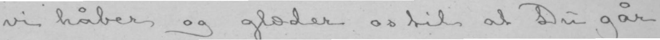vi håber og glæder os til at Du går
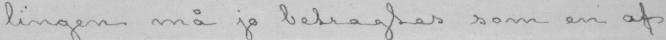lingen må jo betragtes som en af
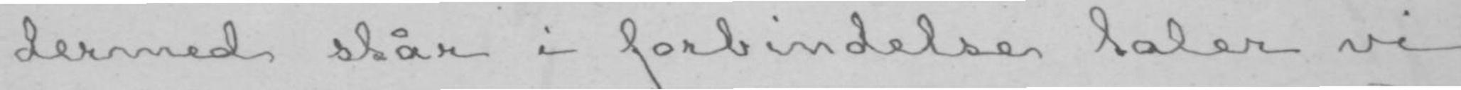dermed står i forbindelse taler vi
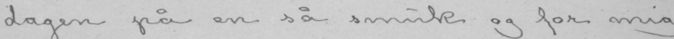dagen på en så smuk og for mig
